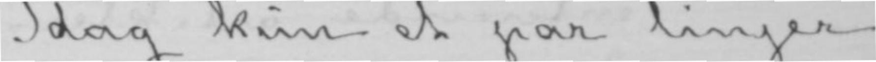Idag kun et par linjer
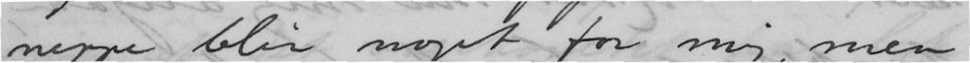neppe blir noget for mig, men
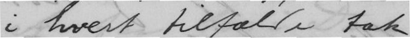i hvert tilfælde tak
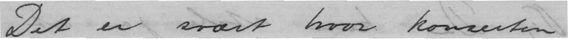Det er svært hvor konserten
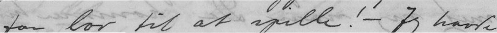faa lov til at spille! - Jeg havde
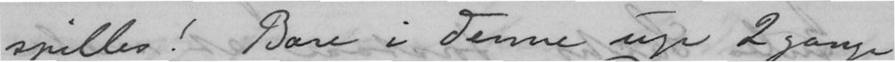spildes! Bare i denne uge 2 gange
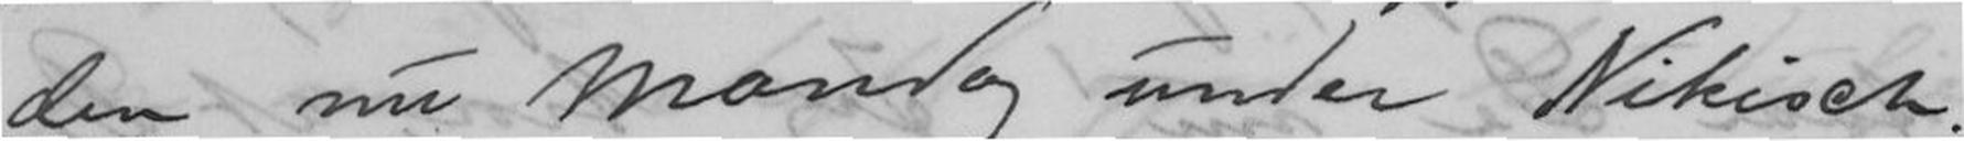den nu Mandag under Nikisch.
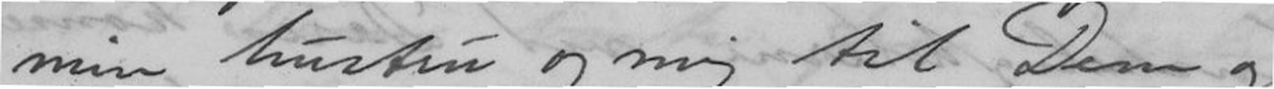min hustru og mig til Dem og
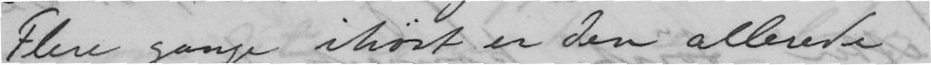Flere gange ihøist er den allerede
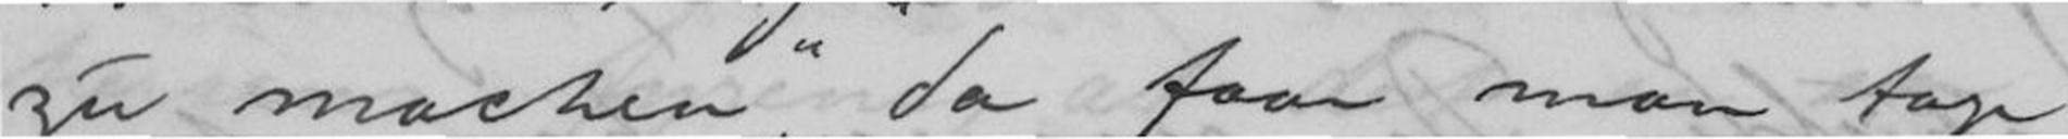zu machen", da faar man tage
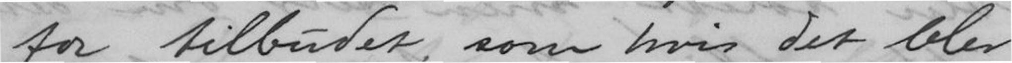for tilbudet, som hvis det blir
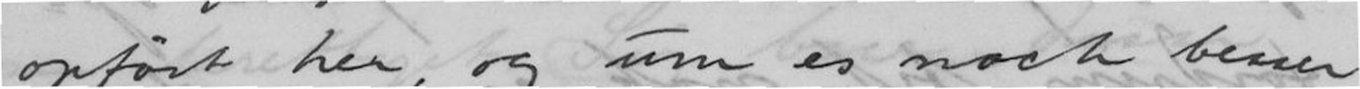opført her, og "um es noch besser
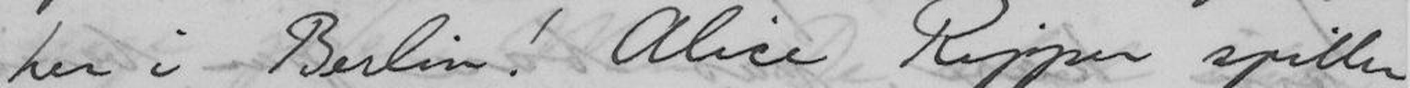her i Berlin! Alice Ripper spiller
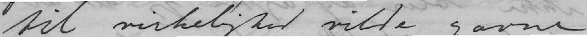til virkelighed vilde gavne
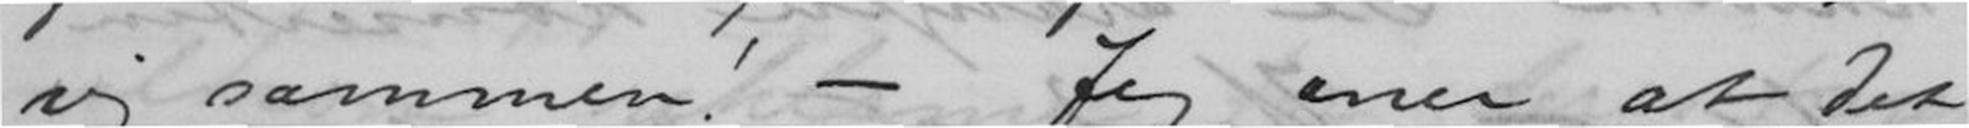sig sammen! - Jeg aner at det
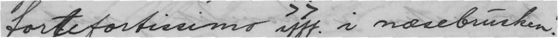fortefortissimo afff. i næsebrusken!
